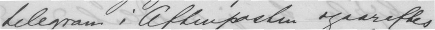telegram i Aftenposten igaaraftes
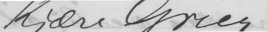Kjære Grieg!
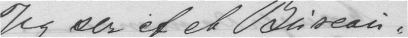Jeg ser af et Bliseau-
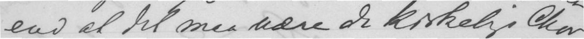end at det maa være de kirkelige Chov-
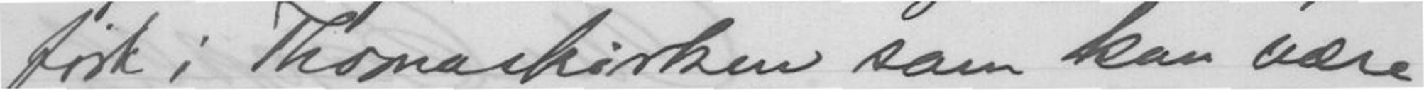ført i Thomaskirken som kan være
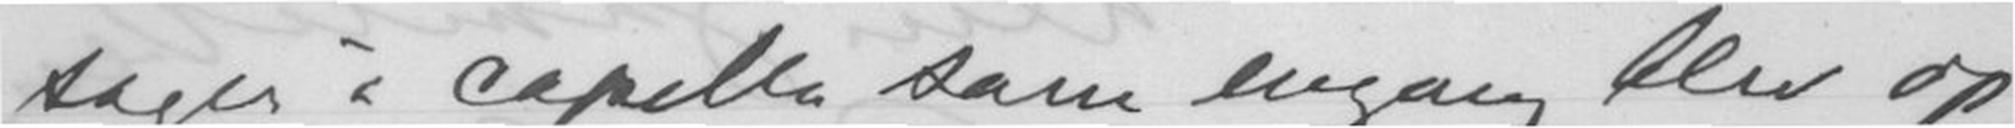sager i capella som engang blev op-
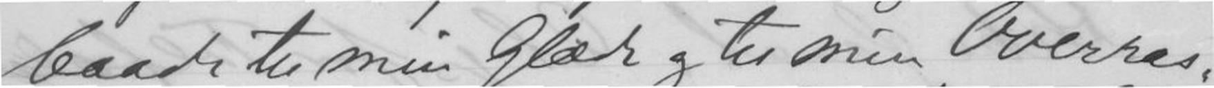baade til min Glæde og til min Overras-
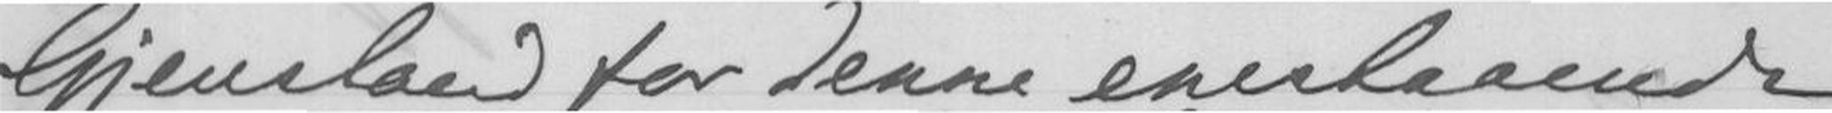Gjenstand for denne enestaaende
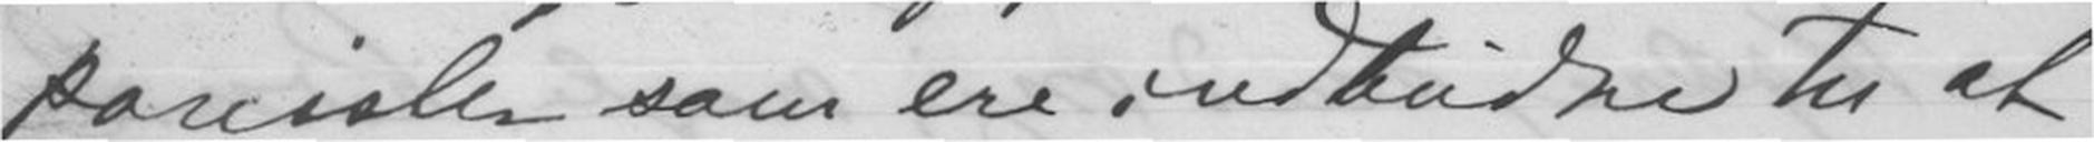ponister som ere indbudne til at
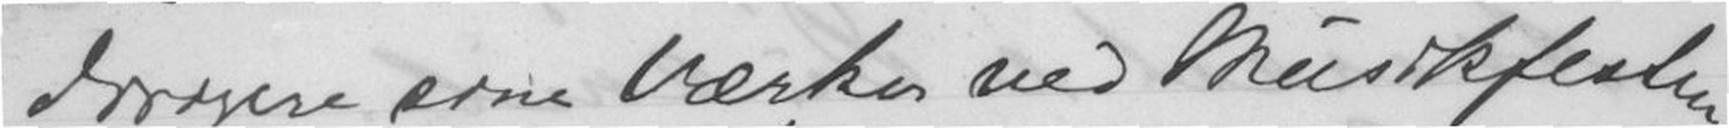dirigere sine Værker ved Musikfesten
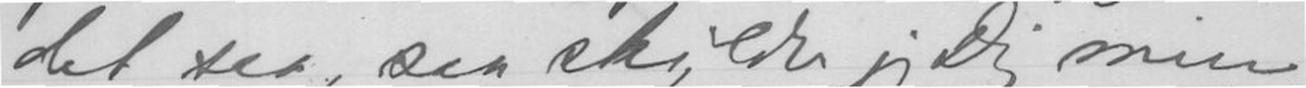det saa, saa skylder jeg Dig min
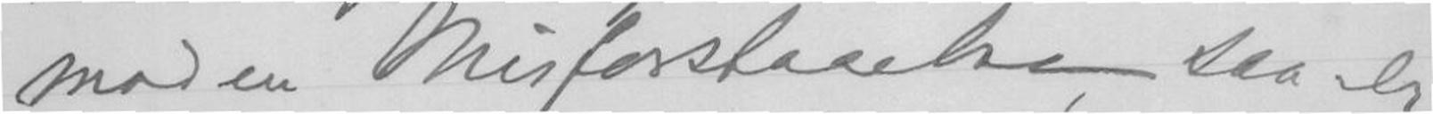med en Misforstaaelse, saa er
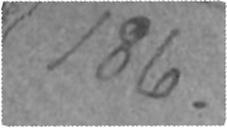186.
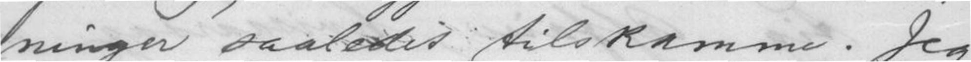ninger saaledes tilskamme. Jeg
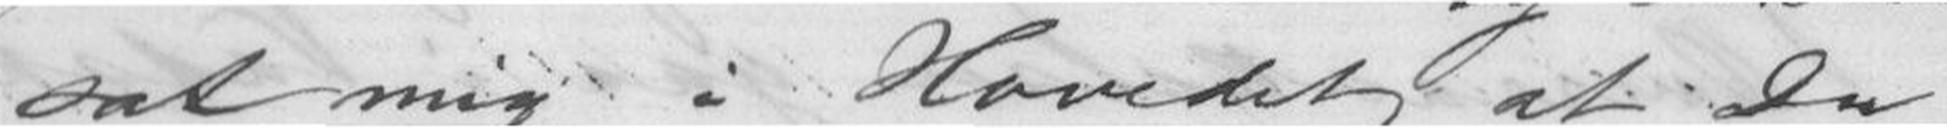sat mig i Hovedet, at Du
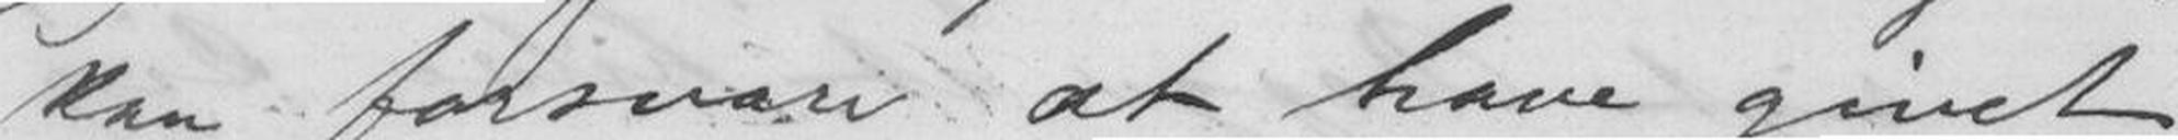kan forsvare at have givet
